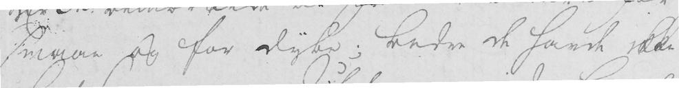smaae og for dybe; bedre de havde ikke
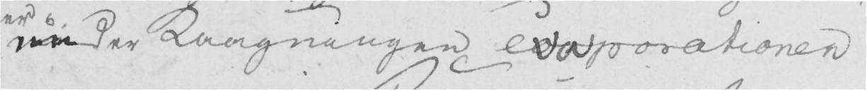under Kaagningen evaporationen
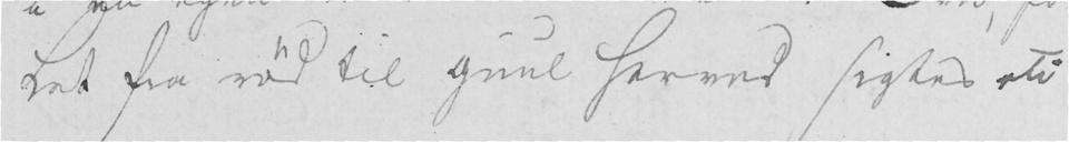let fra rød til guul herved sigtes etc
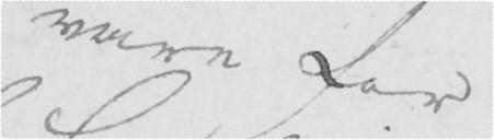vare for
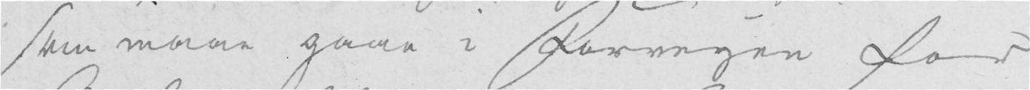som maae gaae i Forveyen for
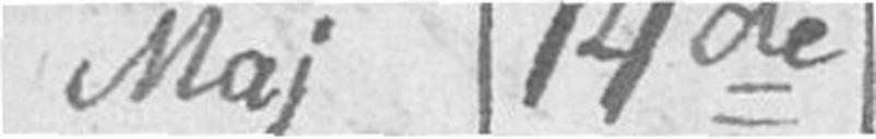Maj 14de
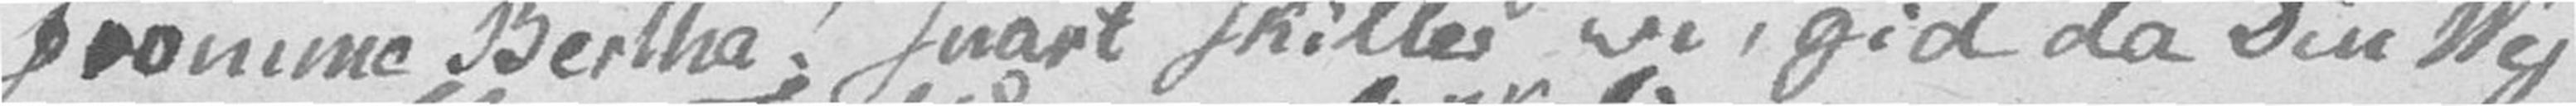fromme Bertha! snart skilles vi, gid da Din Vej
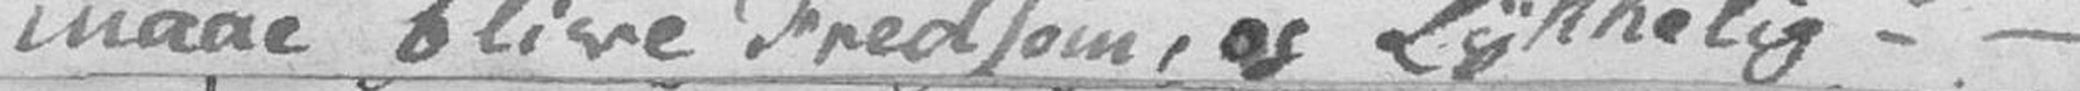maae blive Fredsom, og Lykkelig. –
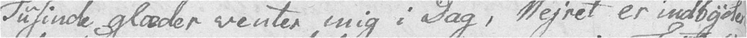Tusinde glæder venter mig i Dag, Vejret er indbyden
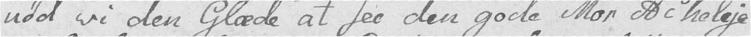nød vi den Glæde at see den gode Mor Acheleje
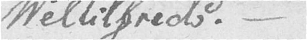Veltilfreds. –
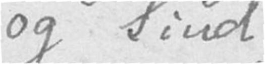og Sind
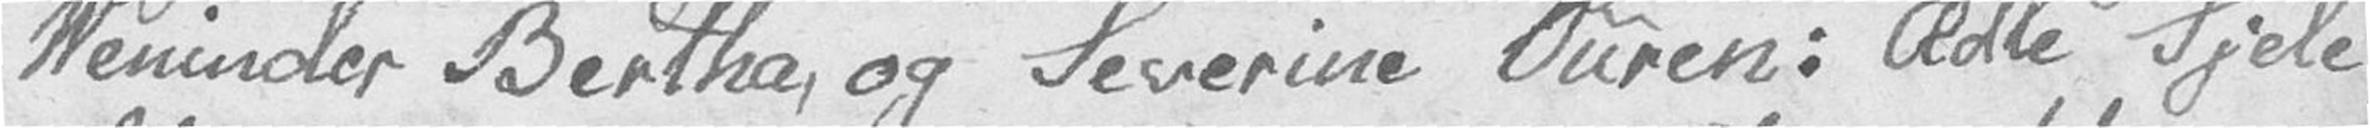Veninder Bertha, og Severine Ouren: Ædle Sjele
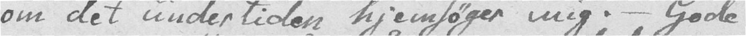om det undertiden hjemsøger mig. – Gode
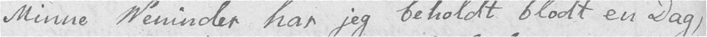Minne Veninder har jeg beholdt blodt en Dag,
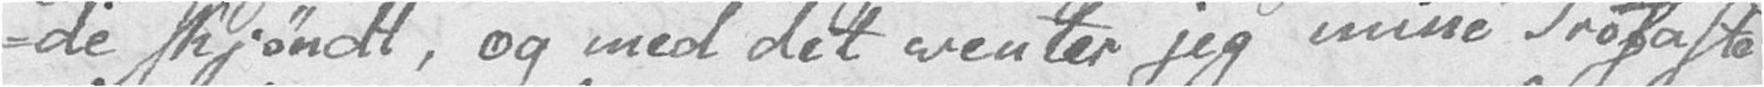-de skjøndt, og med det venter jeg mine Trofaste
